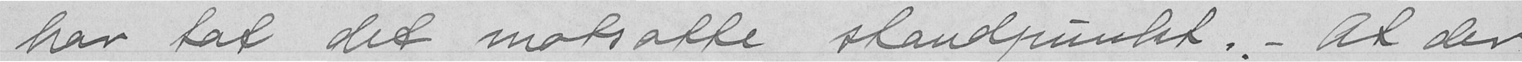har tat det motsatte standpunket.  - At der
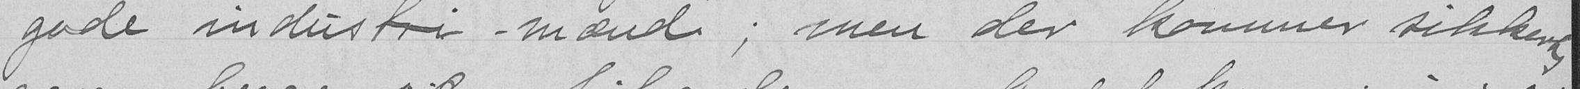gode industri-mænd; men der kommer sikkerlig
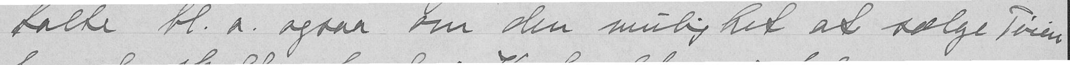talte bl.a. ogsaa om den mulighet at sælge Tøien
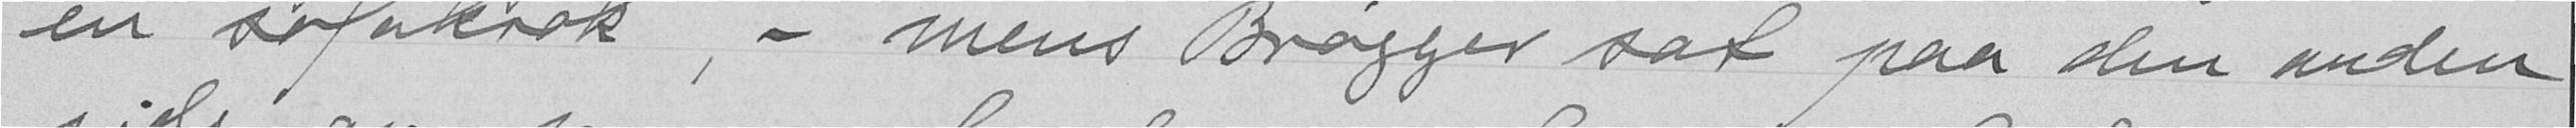en Sofakrok, - mens Brøgger sat paa den anden
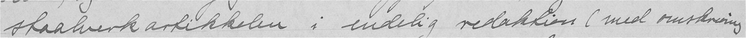staalverkartikkelen i endelig redaktion (med omskriving
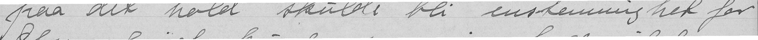paa det hold skulde bli enstemmighet for
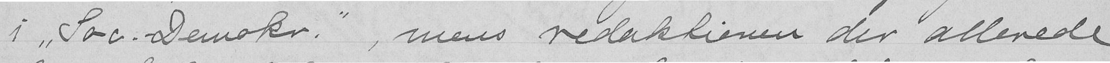i Soc. Demokr., mens redaktionen der allerede
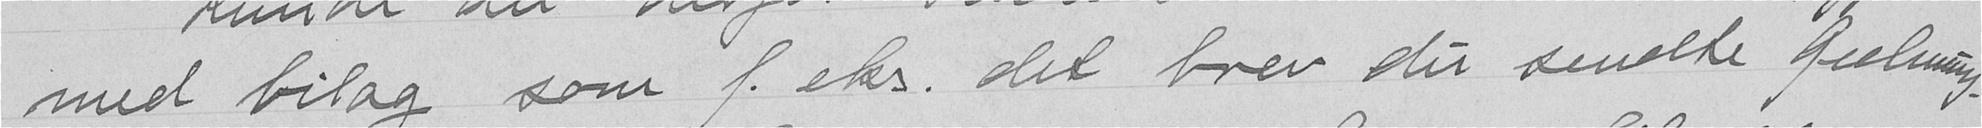med bilag som f. eks. det brev de sendte Geelmuy-
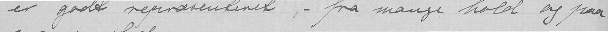er godt repræsenteret, - fra mange hold og paa
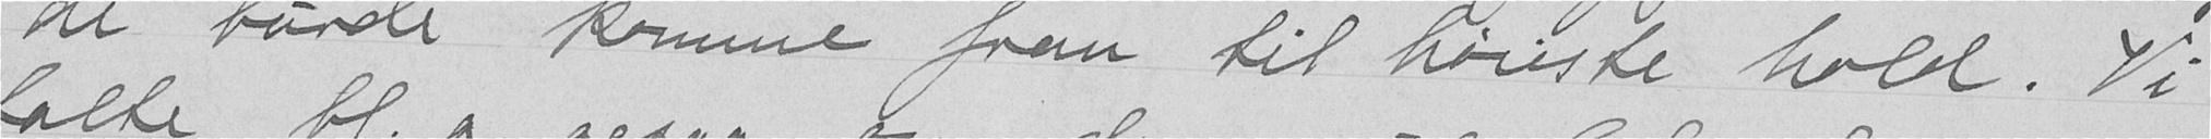de burde komme frem til høieste hold. Vi
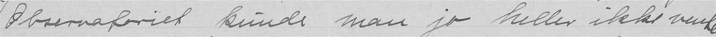Observatoriet kunde man jo heller ikke vente
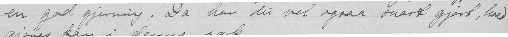en god gjerning. Da har du vel ogsaa snart gjort, hvad
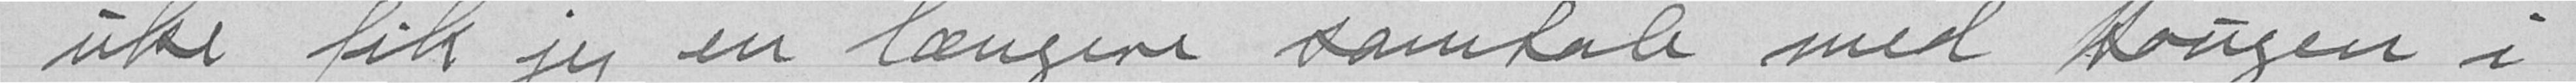uke fik jeg en længere samtale med Kongen i
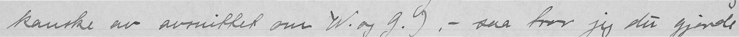kanske av avsnittet om W. og G.),- saa tror jeg du gjorde
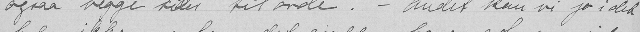ogsaa begge sider til orde. - Andet kan vi jo i det
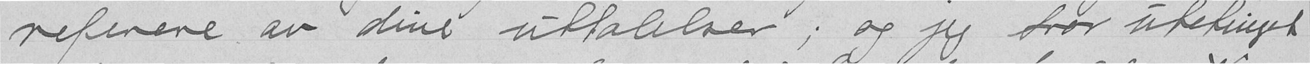referere av dine udtalelser; og jeg tror ubetinget
